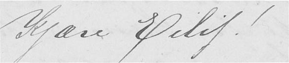Kjære Eilif!
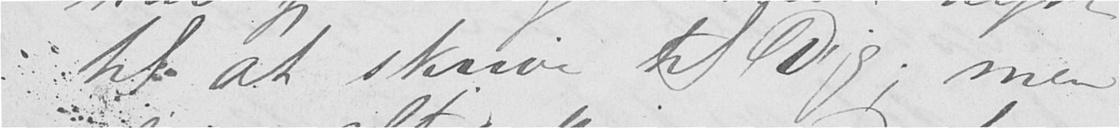til at skrive til Dig; men
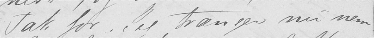Tak for. Jeg trænger nu nem-
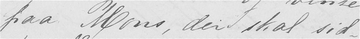paa Mons, der skal sid-
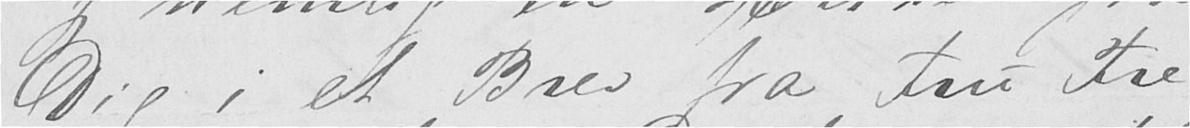Dig i et Brev fra Fru Fre-
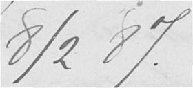8/2 87
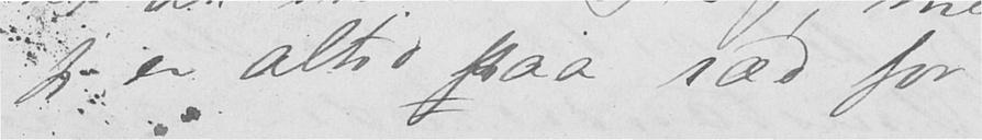jeg er altid saa ræd for
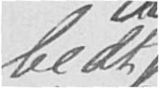bedt
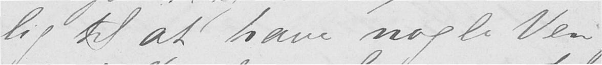lig til at have nogle Ven-
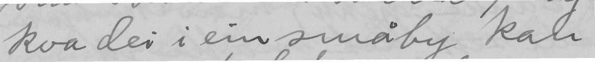kva dei i ein småby kan
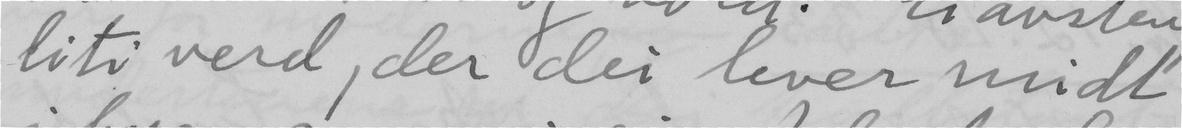liti verd, der dei rever midt
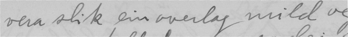vera slik ei overlag mild og
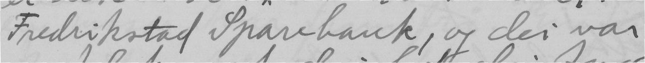Fredrikstad Sparebank, og dei var
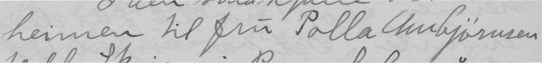heimen til fru Polla Ambjörnsen,
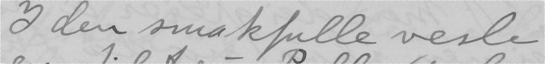I den smakfulle vesle
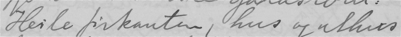Heile firkanten, hus og uthus
